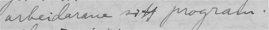arbeidarane sitt program.
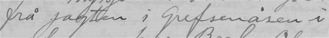frå jagten i Grefsenåsen i
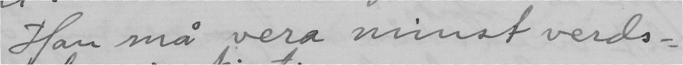Han må vera minst verds-
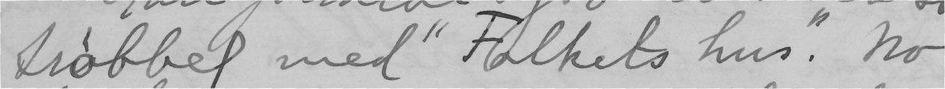tröbbel med "Folkets hus". No
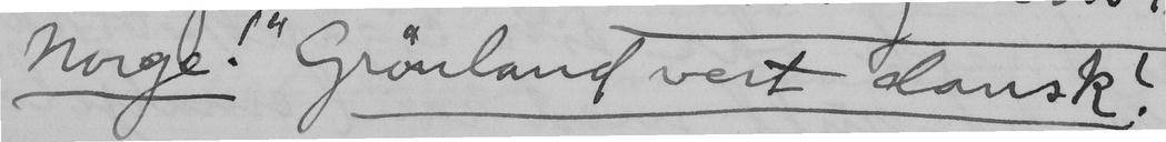Norge!" Grönland vert dansk!
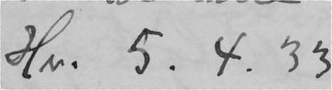Hv. 5.4.33
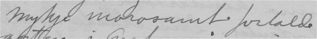Mykje morosamt fortalde
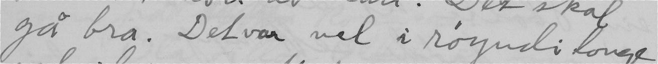gå bra. Det var vel i röyndi longe
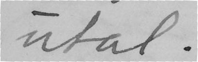utal.
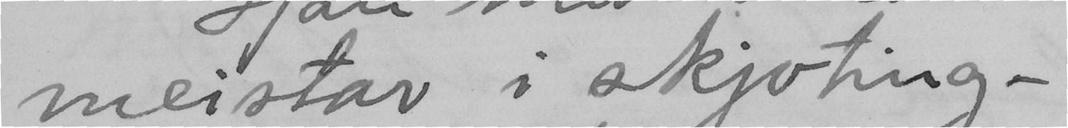meistar i skjoting –
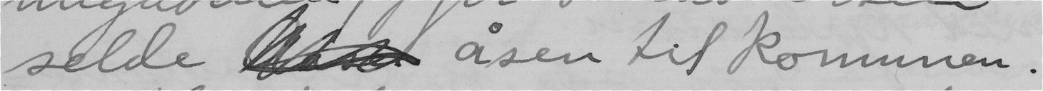selde  åsen til komunen.
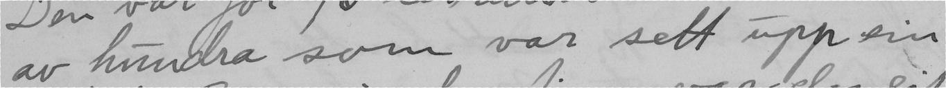av hundra som var sett upp ein
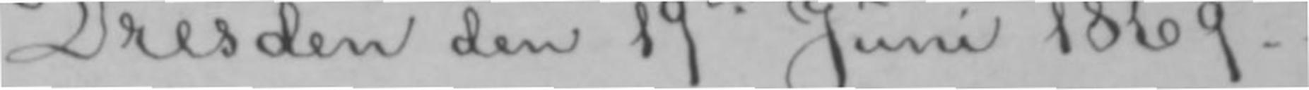Dresden den 19de Juni 1869.-
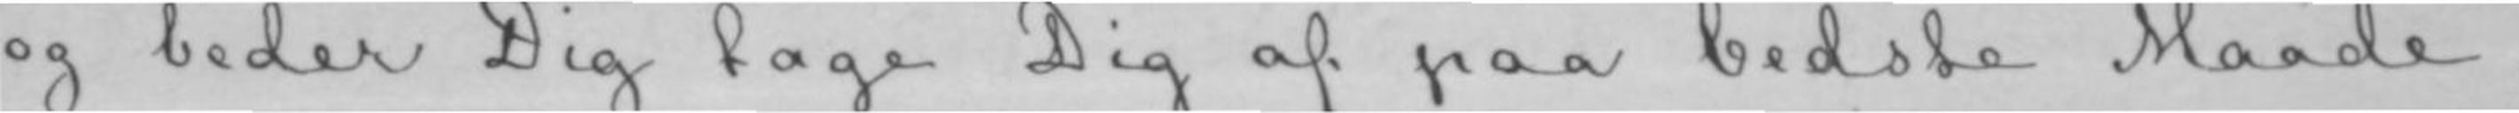og beder Dig tage Dig af paa bedste Maade.
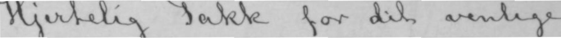Hjertelig Takk for dit venlige
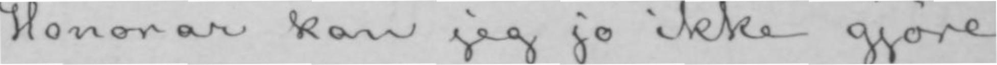Honorar kan jeg jo ikke gjøre
